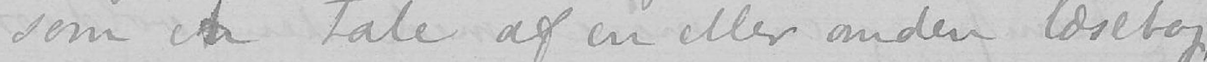som en tale af en eller anden læsebog,
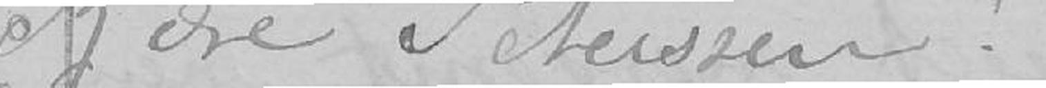Kjære Peterssen!
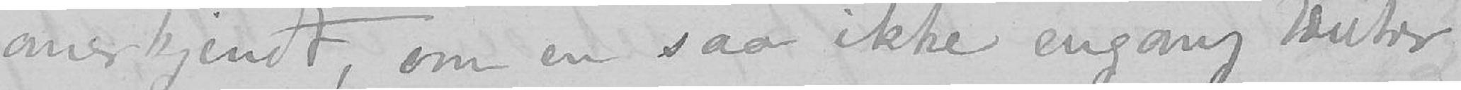anerkjendt, om en saa ikke engang tænker
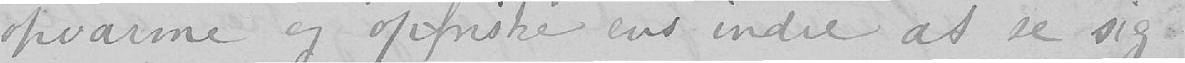opvarme og opfriske ens indre at se sig
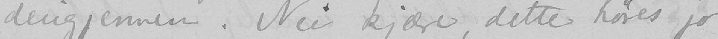derigjennem. Nei kjære, dette høres jo
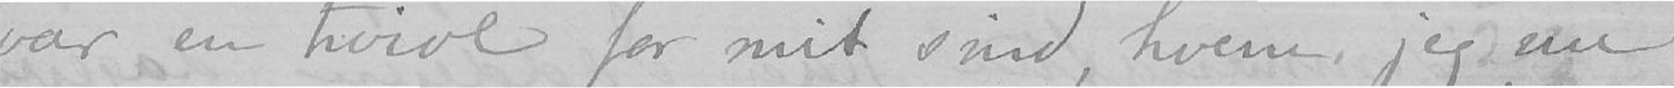var en tvivl for mit sind, hvem jeg nu
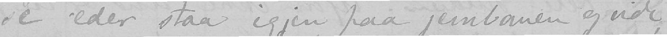se eder staa igjen paa jernbanen og vide,
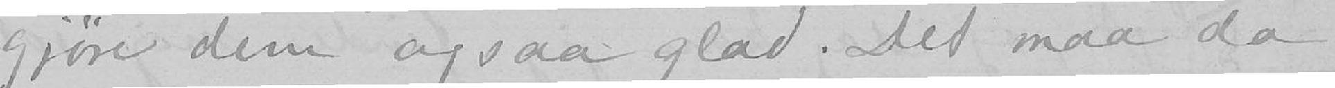vilde gjøre dem ogsaa glad. Det maa da
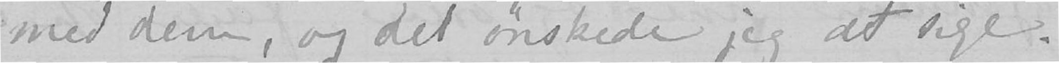med dem, og det ønskede jeg at sige.
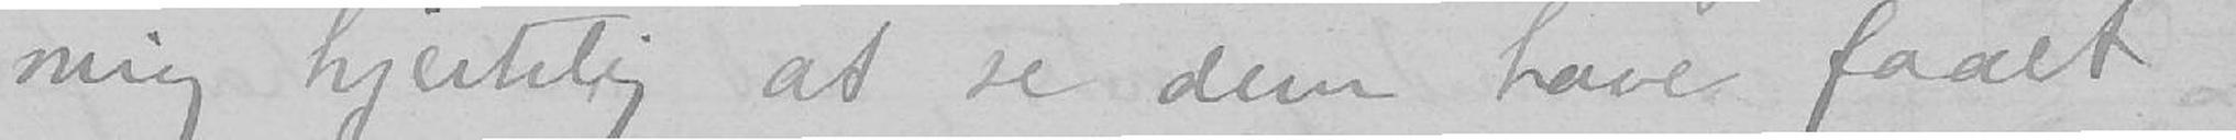mig hjertelig at se dem have faaet
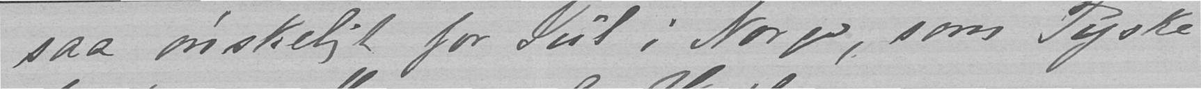saa ønskeligt for Jul i Norge, som Tyske-
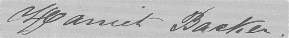Harriet Backer.
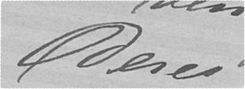Deres
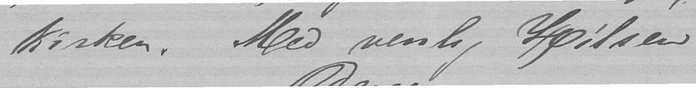kirken. Med venlig Hilsen
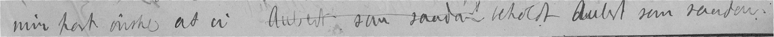min part ønske at vi Aubert som saadan beholdt Aubert som saadan.
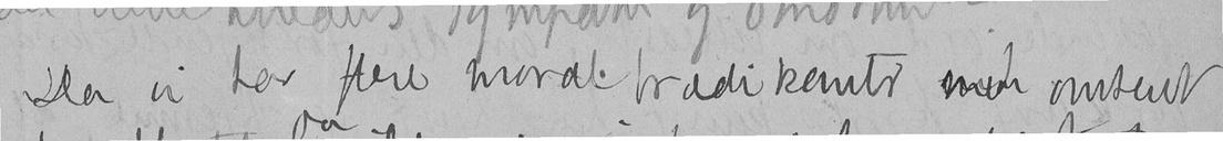Da vi har flere moralprædikanter men omtrent
